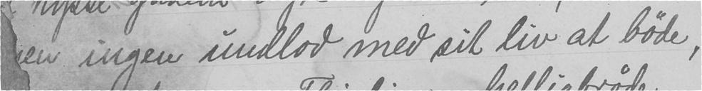en ingen undlod med sit liv at bøde,
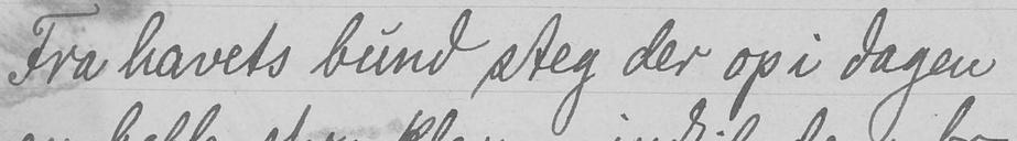Fra havets bund steg der opi dagen
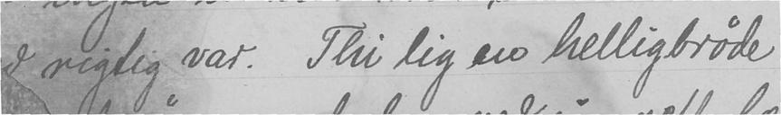d riglig var. Thi lig en helligbrøde
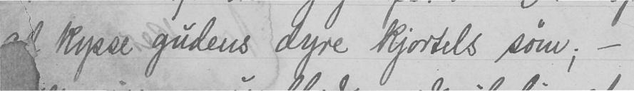at kysse gudens dyre kjortels søm; -
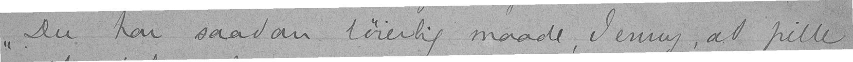"Du har saadan løierlig maade, Jenny, at pille
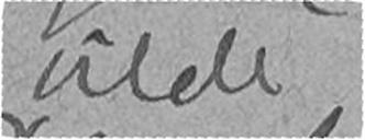vilde
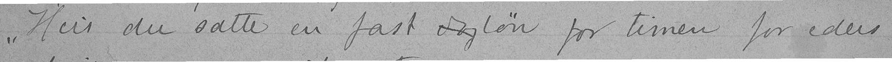"Hvis du satte en fast dagløn for timen for eders
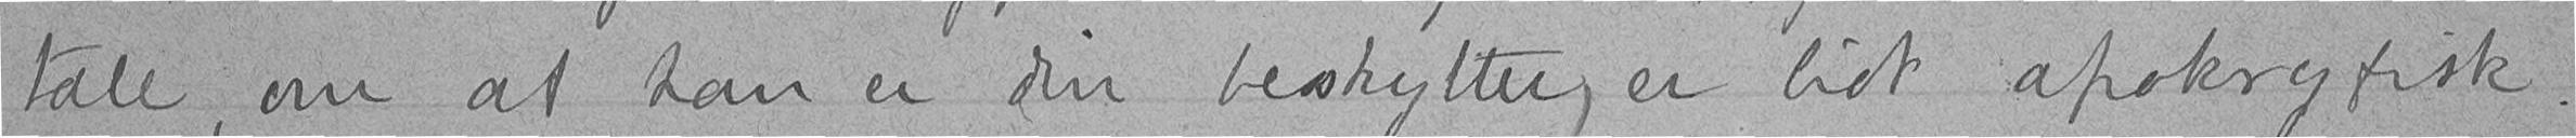tale, om at han er din beskytter, er lidt apokryfisk.
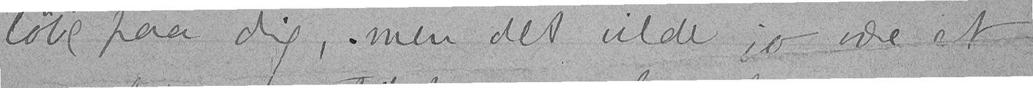løbe paa dig, men det vilde jo være et
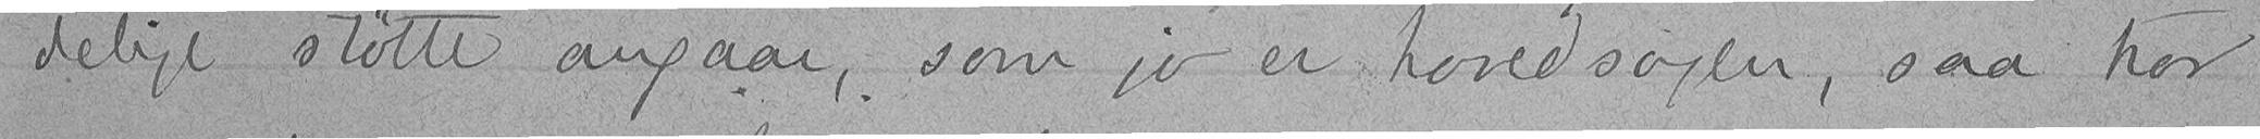delige støtte angaar, som jo er hovedsagen, saa tror
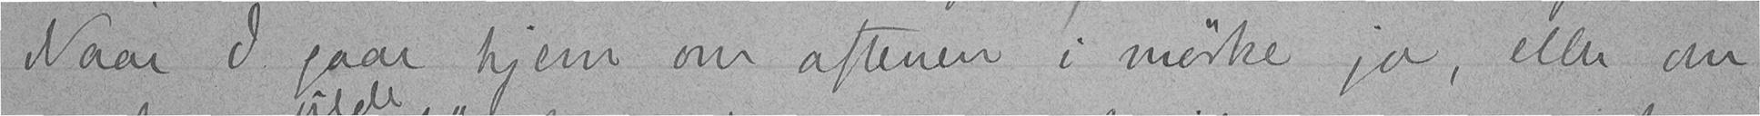Naar I gaar hjem om aftenen i mørke ja, eller om
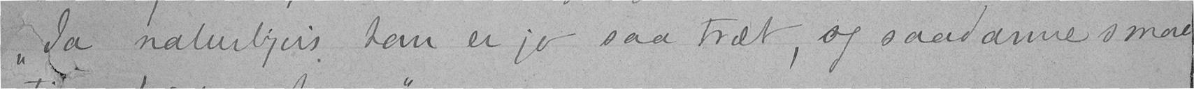"Ja naturligvis, han er jo saa træt, og saadanne smaa
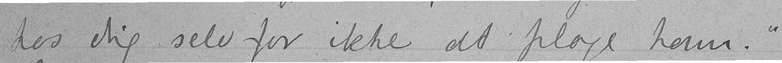hos dig selv for ikke at plage ham."
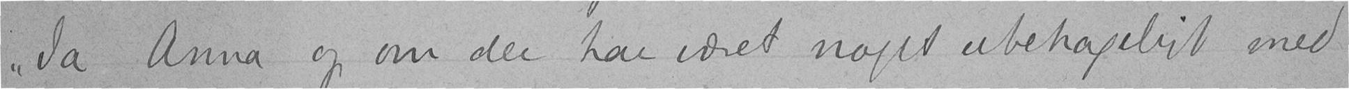"Ja Anna og om der har været noget ubehageligt med
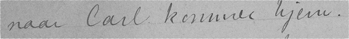naar Carl kommer hjem.
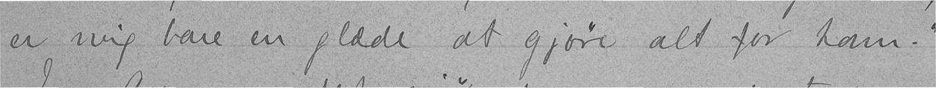er mig bare en glæde at gjøre alt for ham."
